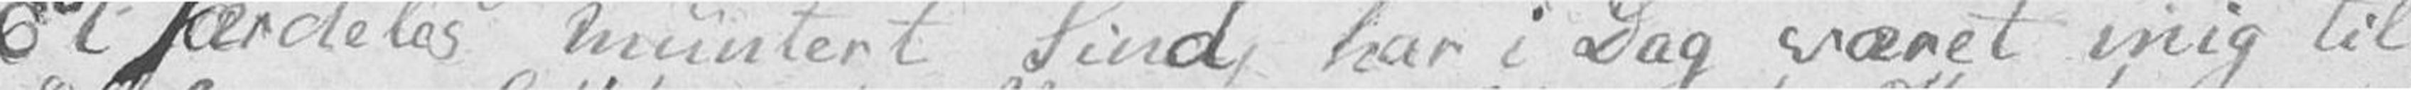Et særdeles muntert Sind, har i Dag været mig til
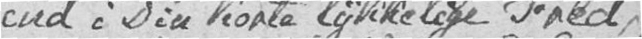ind i Din korte lykkelige Fred,
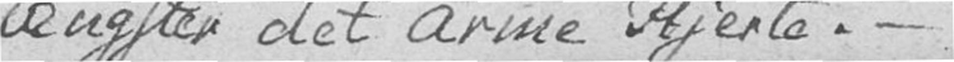ængster det arme Hjerte. –
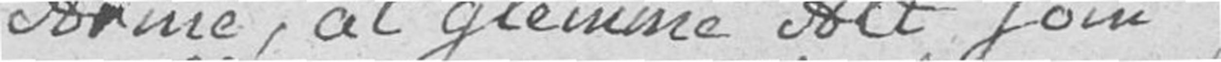Arme, at glemme Alt som
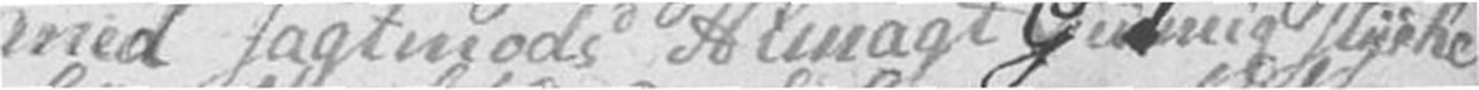med sagtmods Almagt Gud mig styrke
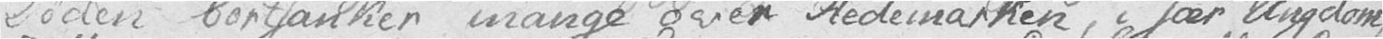Døden bortsanker mange over Hedemarken, i sær Ungdom.
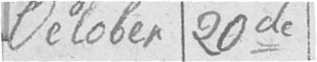October 20de
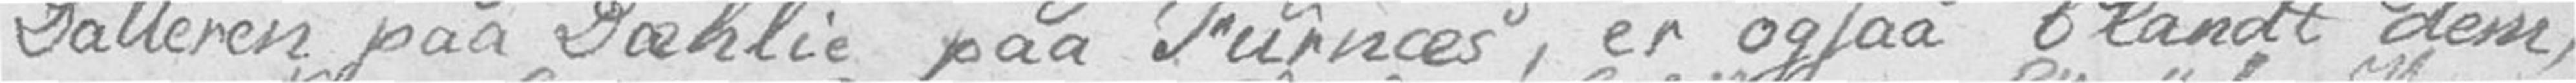Datteren paa Dæhlie paa Furnæs, er også blant dem,
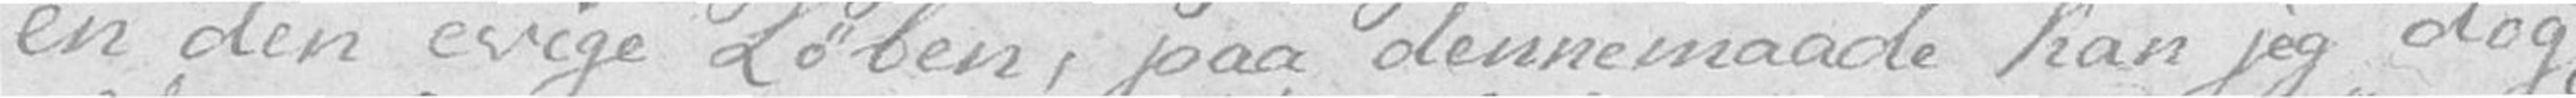en den evige Løben, paa dennemaade kan jeg dog
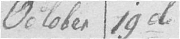October 19de
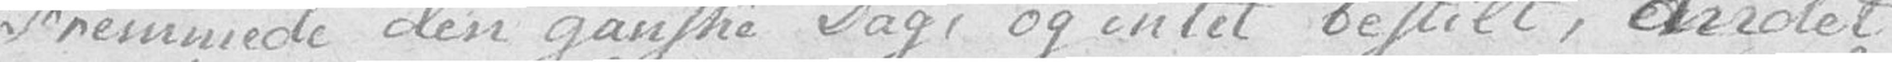Fremmede den ganske Dag, og intet bestilt, andet
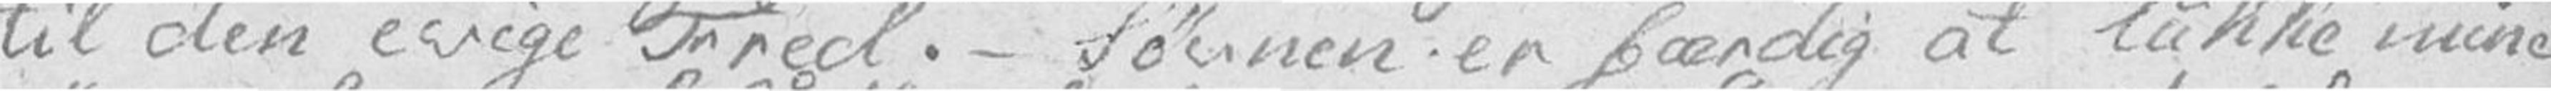til den evige Fred. – Søvnen er færdig at lukke mine
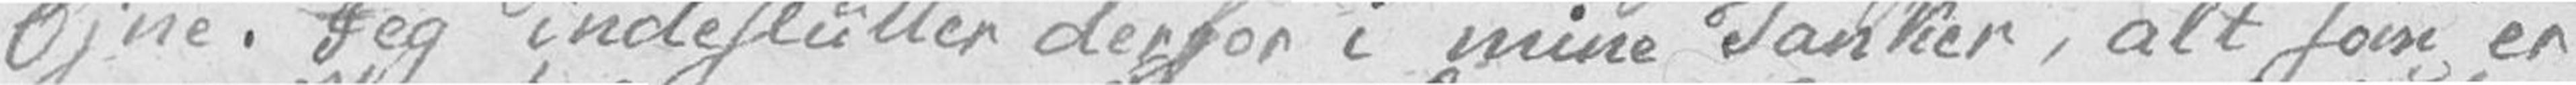Øjne. Jeg indeslutter derfor i mine Tanker, alt som er
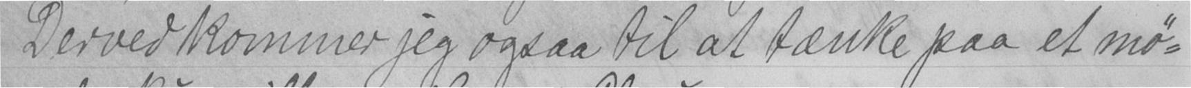Derved kommer jeg ogsaa til at tænke paa et mø-
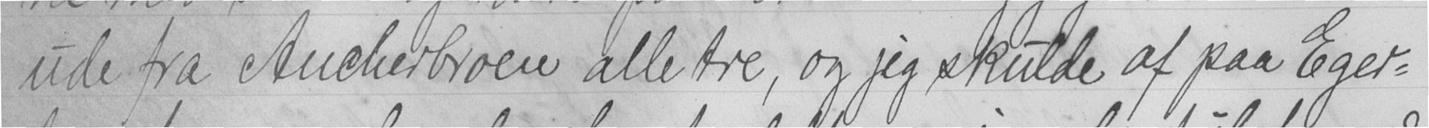ude fra Ancherbroen alle tre, og jeg skulde af paa Eger-
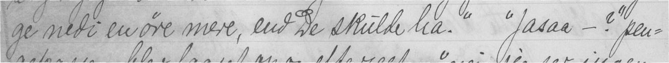ge nedi en øre mere, end de skulde ha."  "Jasaa - ?" pen-
